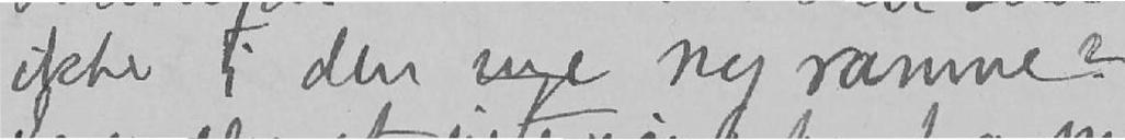ikke i den nye ny ramme?
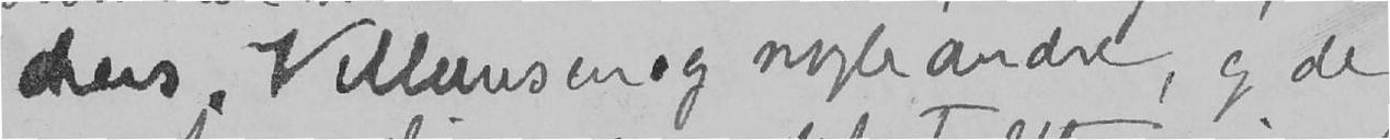ckers, Villumsen og nogle andre, og de
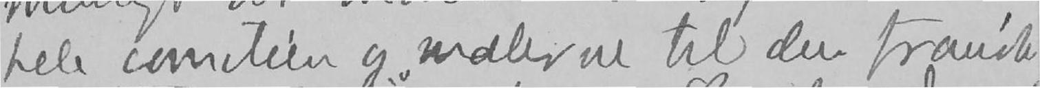hele comitéen og malerne til den franske
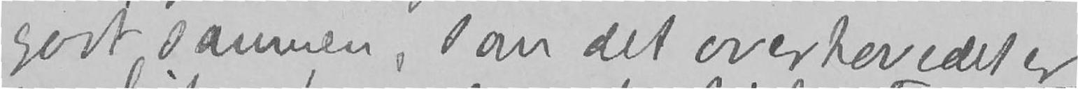godt sammen, som det overhovedet er
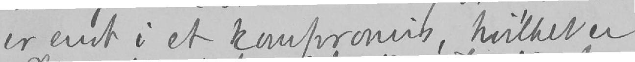er endt i et kompromis, hvilket er
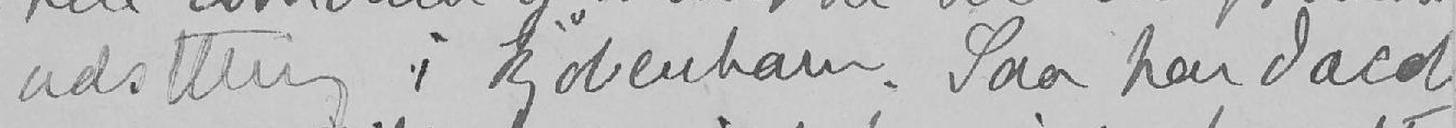udstilling i Kjøbenhavn. Saa har Jacob-
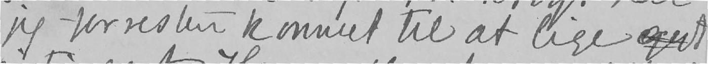jeg forresten kommet til at lige godt
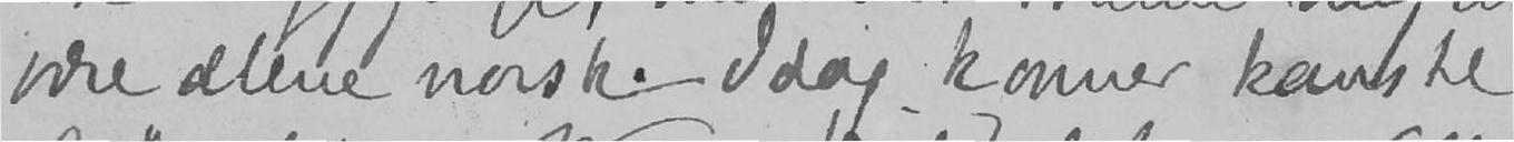være alene norsk. Idag kommer kanske
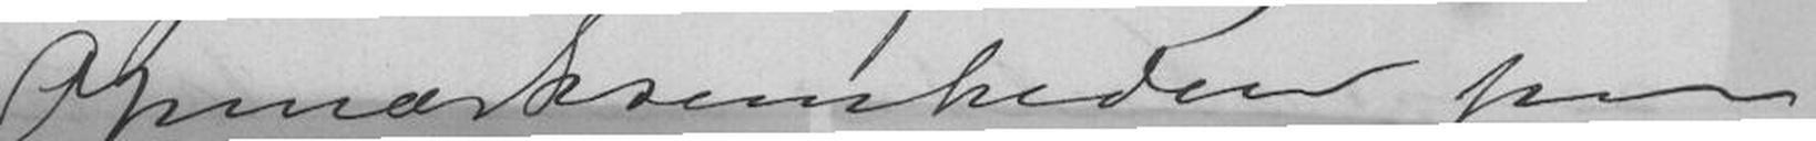Opmærksomheden paa
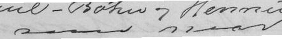som naar
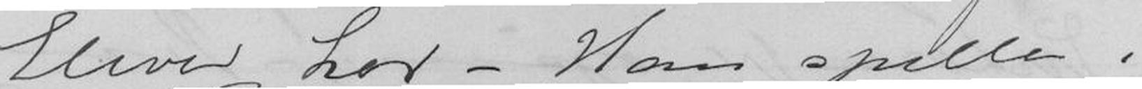Elever her - Han spillede i
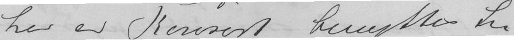her er Konsert benyttes til
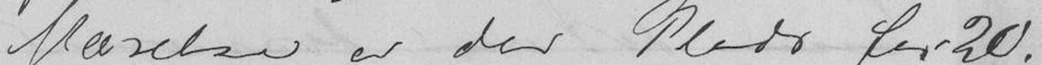Værelse er der Plads til 20.
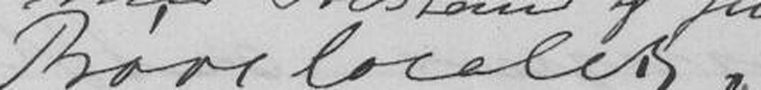med Bestan af Jull-Bøhn og Hennum
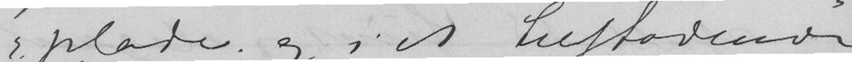pladse og i et tilstødende
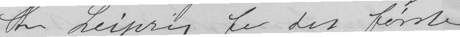til Leipzig for det første
